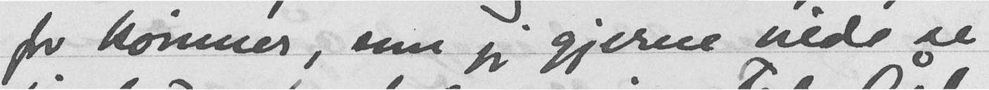for kvinner som jeg gjerne vilde se
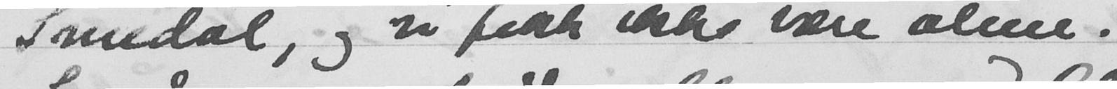Smedal, og vi fikk ikke være alene.
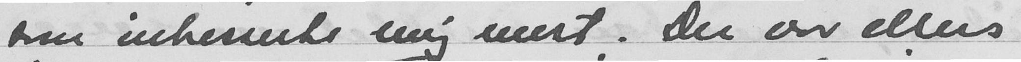som intresserte mig mest. Der var ellers
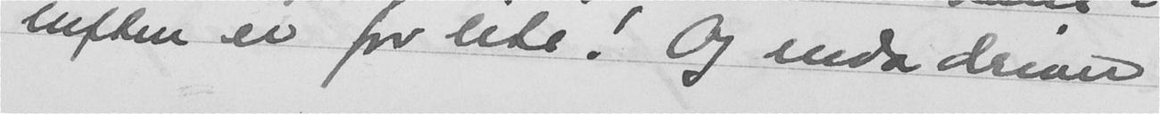luften er forlite. Og enda driver
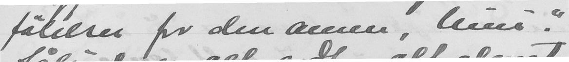følelser for den annen, Nini."
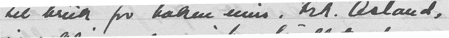til bruk for boken min. Frk. Åsland,
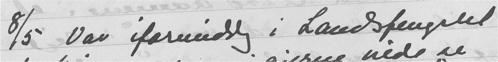8/5 Var iformiddag i Landsfengslet
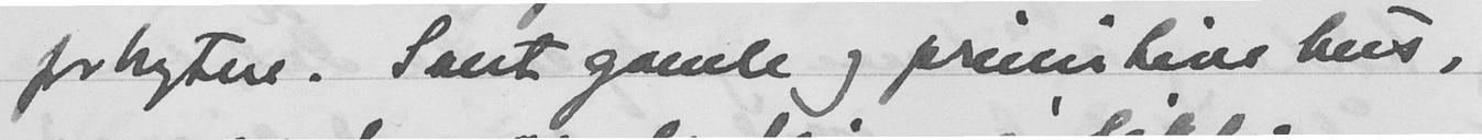forbrytere. Fant gamle og primitive hus,
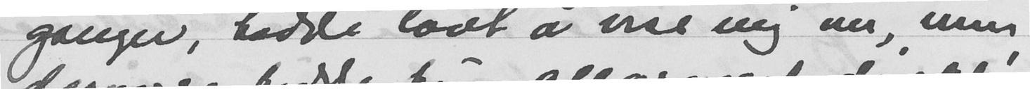ganger, hadde lovt å vise mig om, men
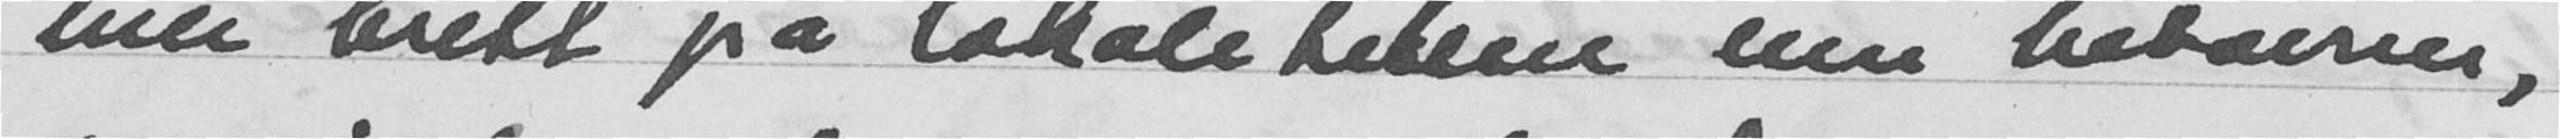mer brett på lokalitetene enn bebsirin,
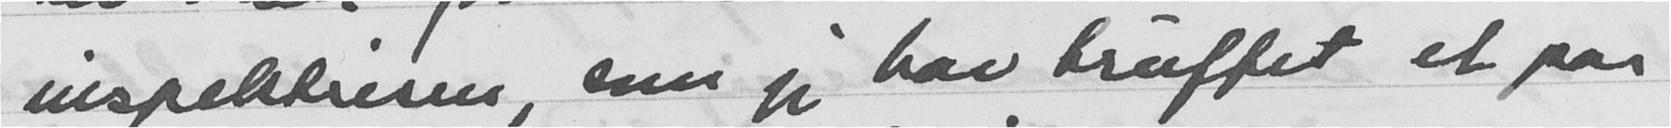inspektrisen, som jeg har truffet et par
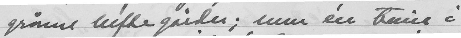grønne luftegårder; men en time i
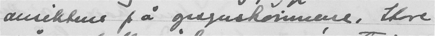ansiktene på opsguskommene. Store
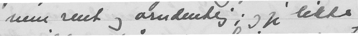men rent og ordentlig; og jeg likte
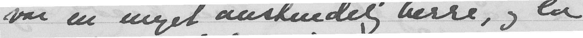var en meget anstendelig herre, og la
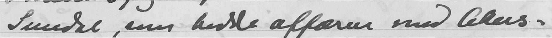Smedal, som hadde affæren med Akers-
